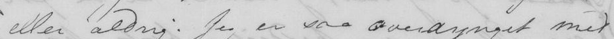eller aldrig. Jeg er saa overdynget med
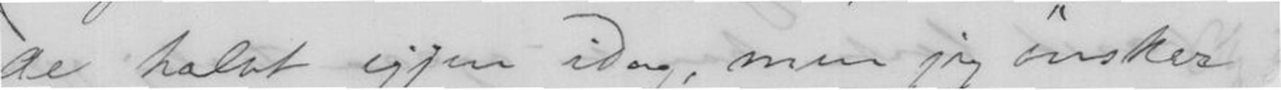de halvt igjen idag, men jeg ønsker
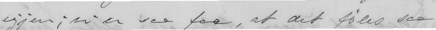igjen; vi er saa faa, at det føles saa
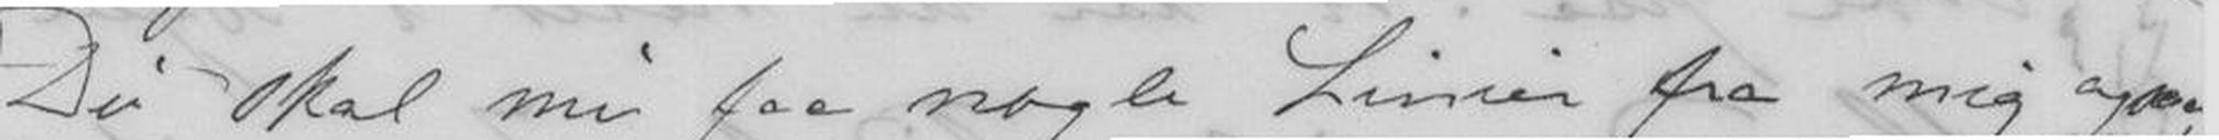Du skal nu faa nogle Linier fra mig og,
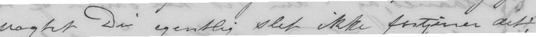uagtet Du egentlig. slet ikke fortjener det;
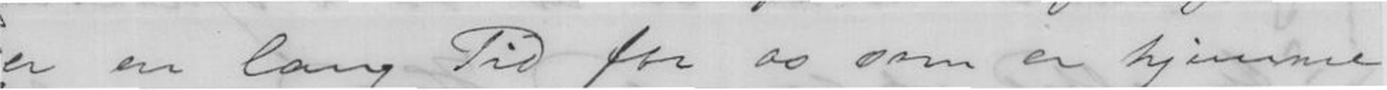er en lang Tid for os som er hjemme
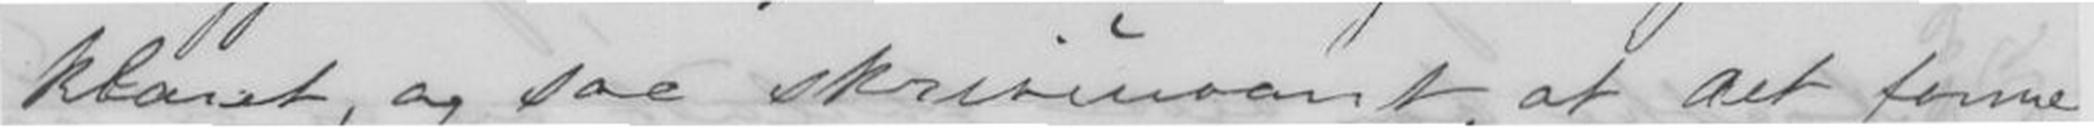klaret, og saa skriveuvant, at det forme-
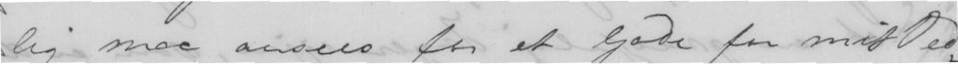lig maa ansees for et Gode for mit Ved-
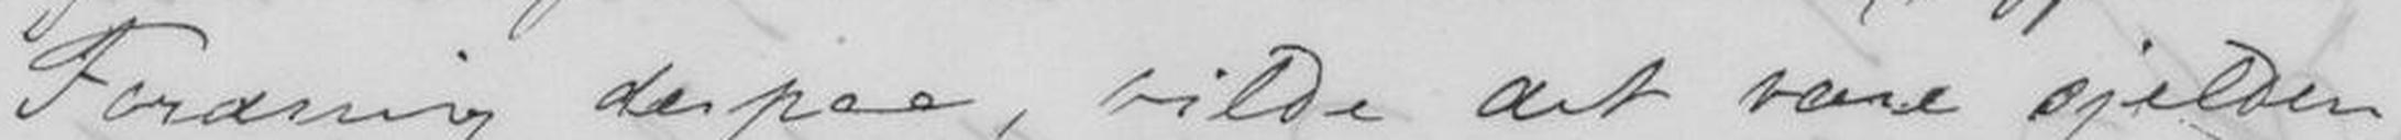Fordring derpaa, vilde det være sjelden
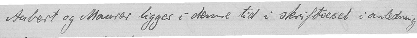Aubert og Maurer ligger i denne tid i skriftvesel i anledning
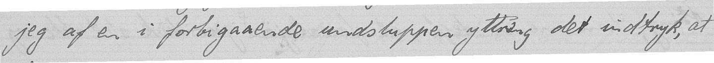jeg af en i forbigaaende undsluppen yttring det indtryk, at
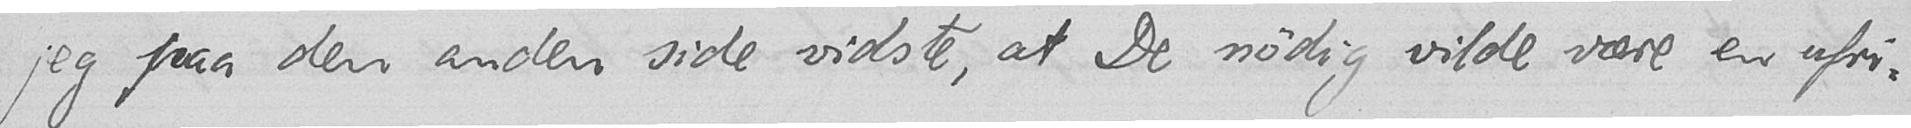jeg paa den anden side vidste, at De nødig vilde være en ufri-
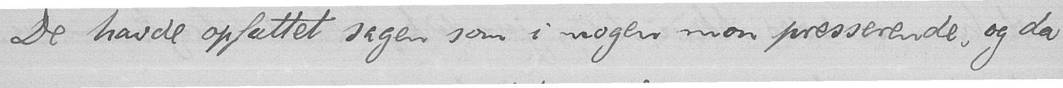De havde opfattet sagen som i nogen mon presserende, og da
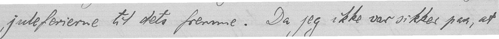juleferierne til dets fremme. Da jeg ikke var sikker paa, at
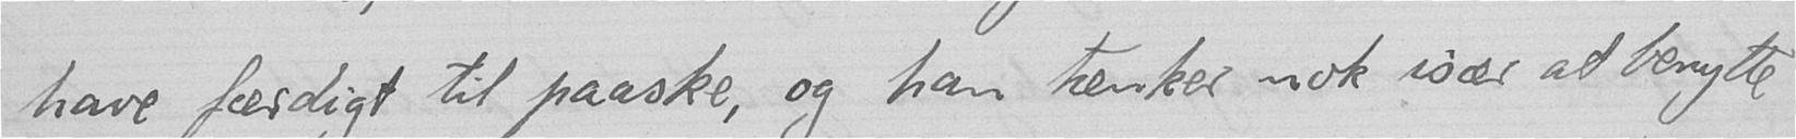have færdigt til paaske, og han tænker nok især at benytte
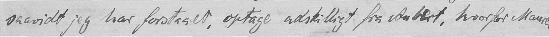saavidt jeg har forstaaet, optage adskilligt for Aubert, hvorfor Maurer
